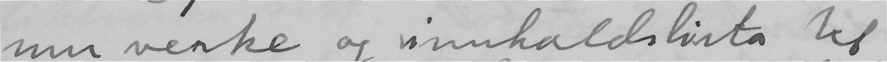um verke og innhaldslista til
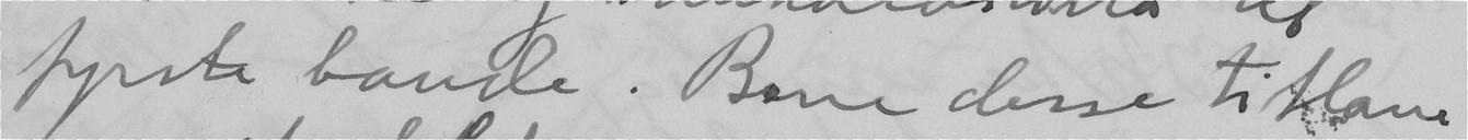fyrste bande. Berre desse titlane
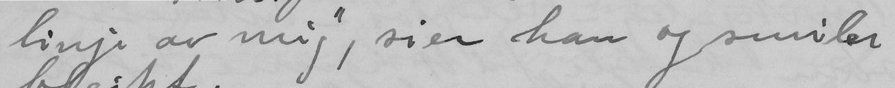linji av mig", sier han og smiler
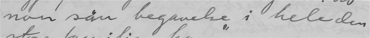non sån begavelse i hele den
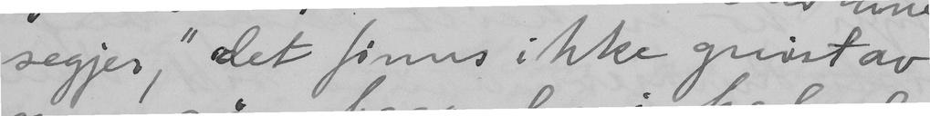segjes, "det finns ikke gnist av
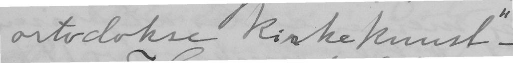ortodokse Kirkekunst" –
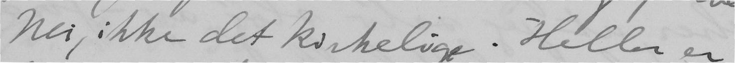Nei, ikke det kirkelige. Heller er
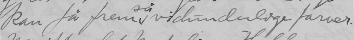kan få frem så vidunderlige farver.
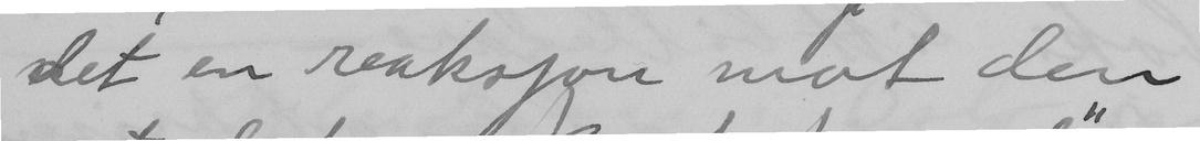det en reaksjon mot den
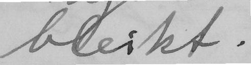bleikt.
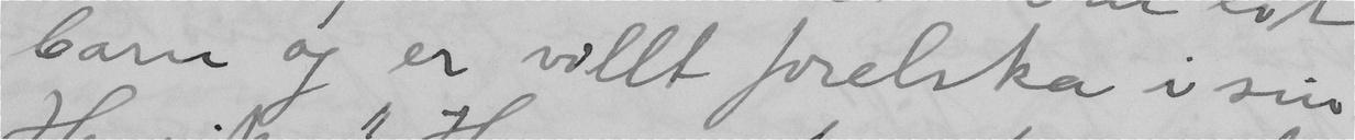barn og er villt forelska i sin
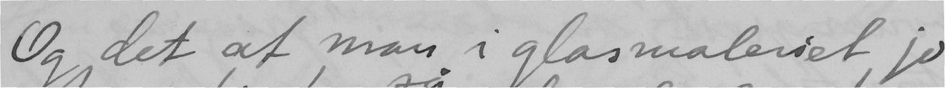Og det at man i glasmaleriet jo
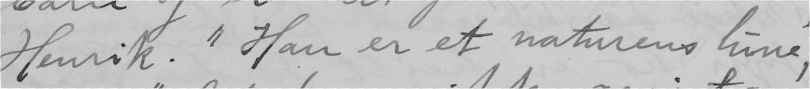Henrik. "Han er et naturens lune",
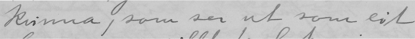kvinna, som ser ut som eit
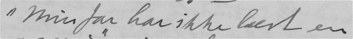"Min far har ikke læst en
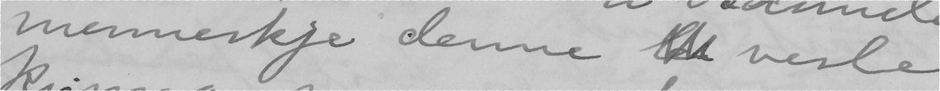menneskje denne vesle
